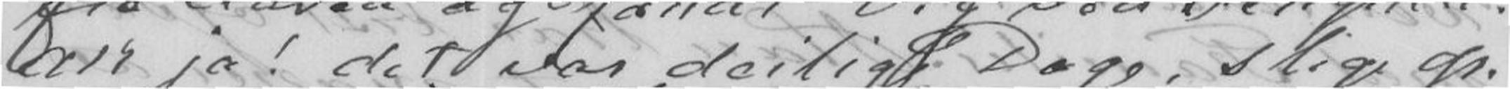Ak ja! det var deilig Dage, slig dr
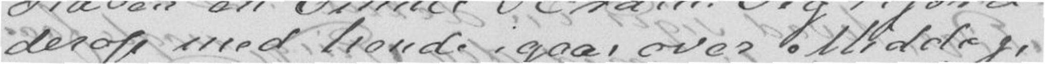derop med hende igaar over Middag,
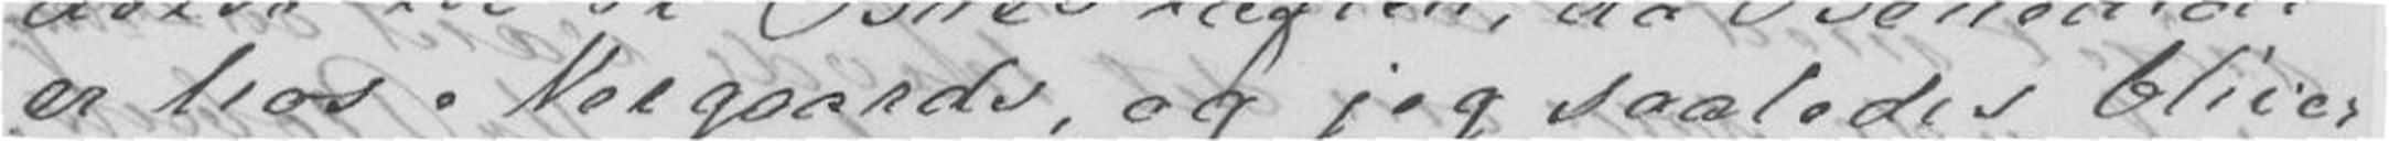er hos Nergaard, og jeg saaledes bliver
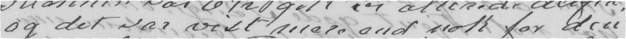og det var vist mere end nok for den
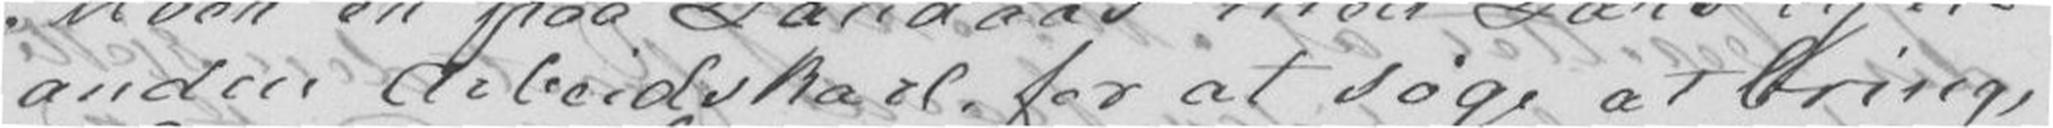anden Arbeidskarl, for at søge at bringe
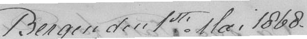Bergen den 1ste Mai 1868.
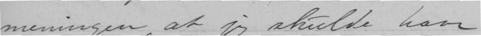meningen, at jeg skulde have
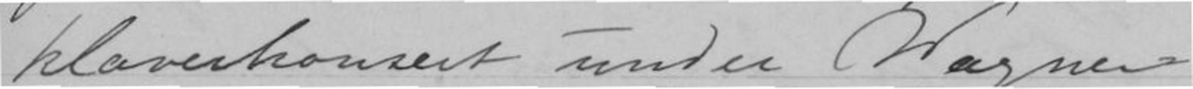klaverkonsert under Wagner
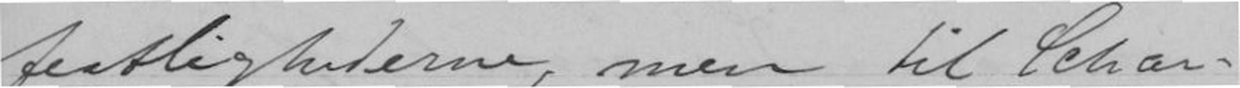festlighederne, men til Schar-
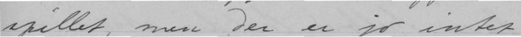spillet, men der er jo intet
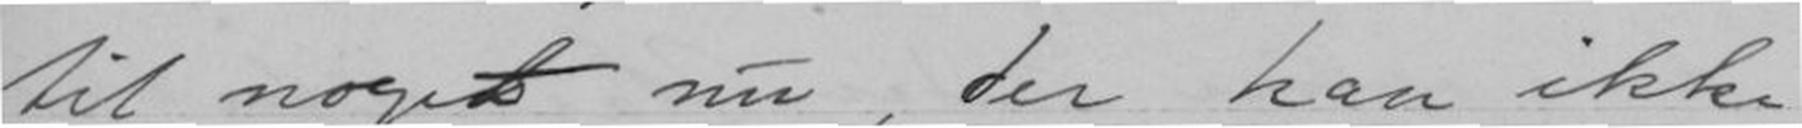til noget nu, der han ikke
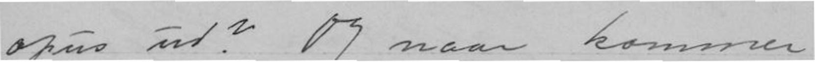opus ud? Og naar kommer
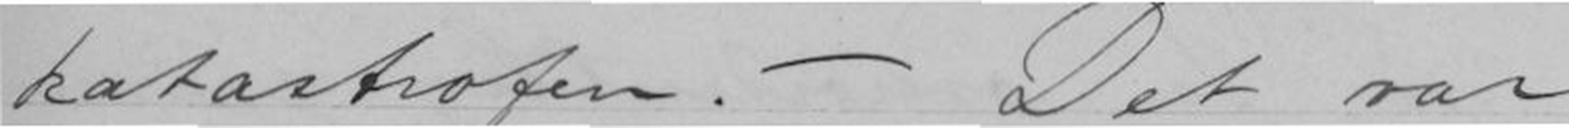katastrofen. - Det var
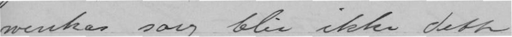wenkas sorg blir ikke dette
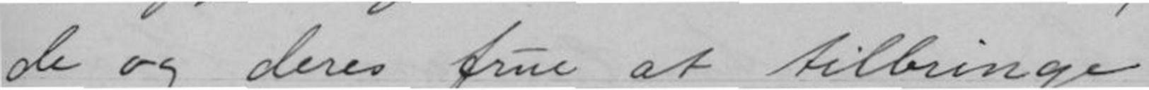de og deres frue at tilbringe
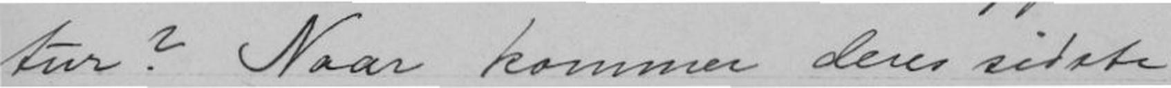tur? Naar kommer deres sidste
